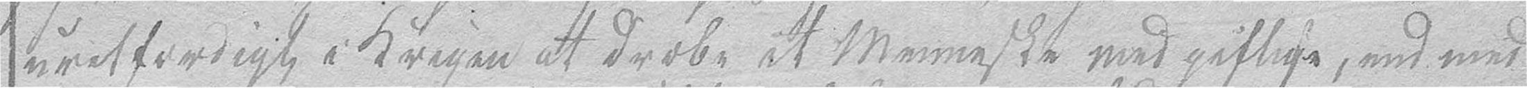uretfærdigt i Krigen at dræbe et Menneske med giftige, end med
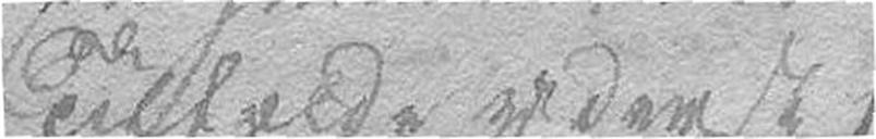Tilfælde yderst
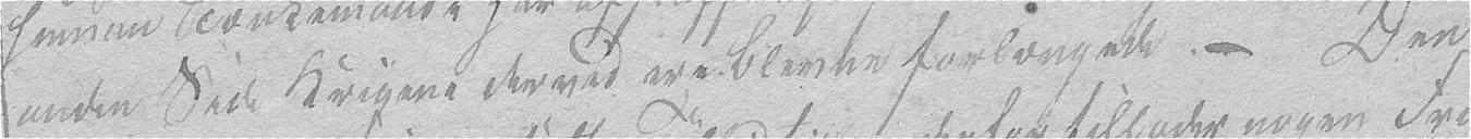anden side Krigene derved ere blevne forlængede. – Den
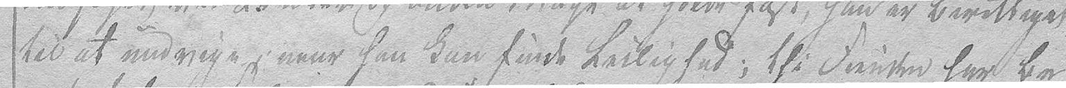til at undvige, naar han kan finde Leilighed; thi Fienden har be-
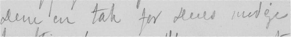Dem en tak for Deres modige
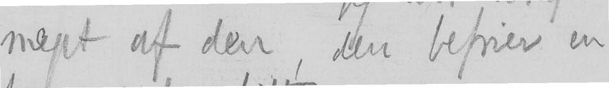meget af den, den befrier en
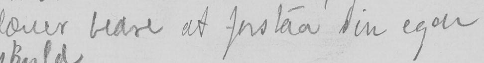lærer bedre at forstaa sin egen
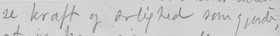se kraft og ærlighed som gjorde,
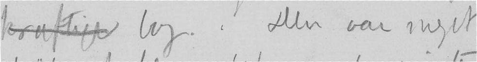kraftige bog. Den var meget
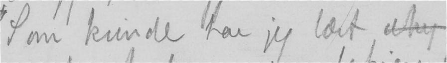Som kvinde har jeg lært uhy
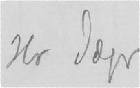Hr Jæger
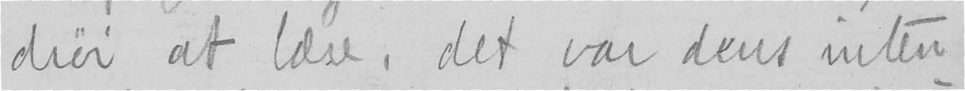drøi at læse, det var dens inten-
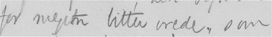for megetn bitter vrede, som
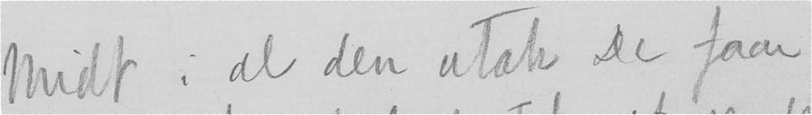Midt i al den utak De faar,
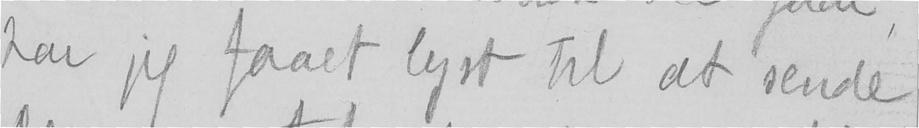har jeg faaet lyst til at sende
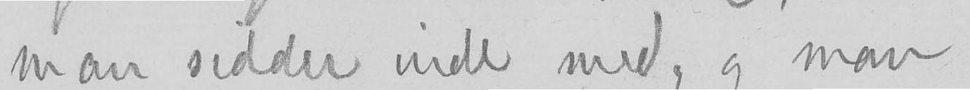man sidder inde med, og man
